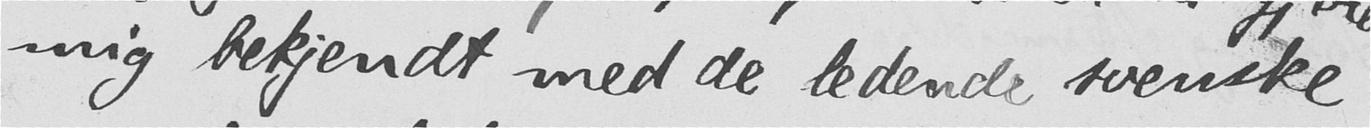mig bekjendt med de ledende svenske
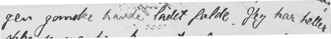gen ganske havde ladet falde. Jeg har heller
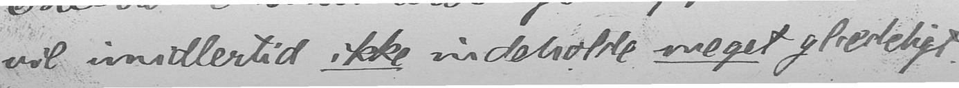vil imidlertid ikke indeholde meget glædeligt.
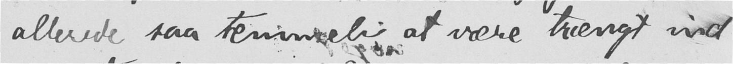allerede saa temmeli at være trængt ind
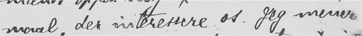maal, der interessere os. Jeg mener
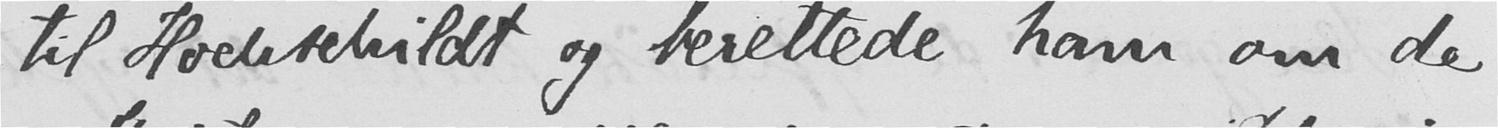til Hochschildt og berettede ham om de
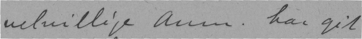velvillige Anm. har git
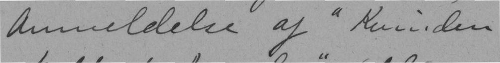anmeldelse af "Kvinden
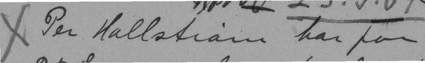x Per Hallstrøm har for
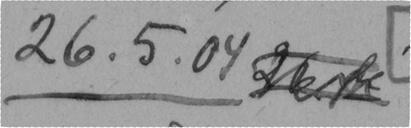26.5.04
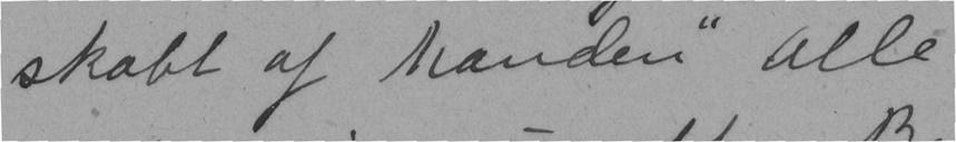skabt af Manden" alle
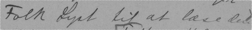Folk Lyst til at læse den
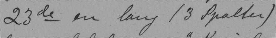23de en lang (3 Spalter)
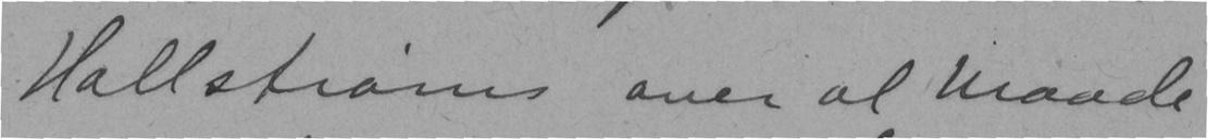Hallstrøms over al maade
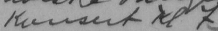Konsert kl 7
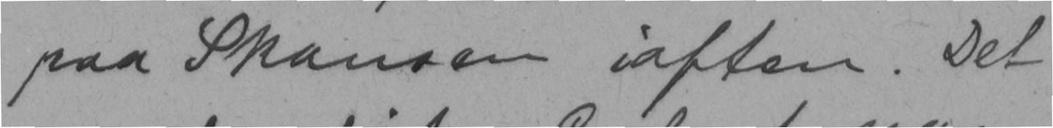paa Skansen iaften. Det
